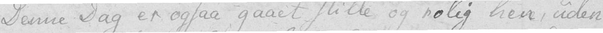Denne Dag er ogsaa gaaet stille og rolig hen, uden
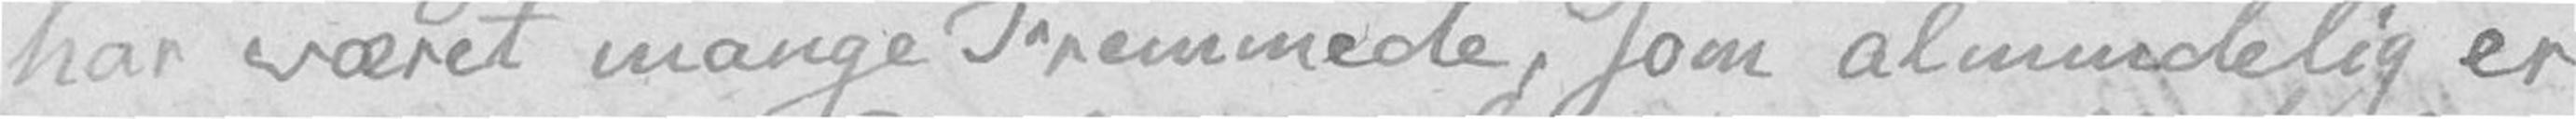har været mange Fremmede, som almindelig er
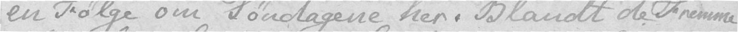en Følge om Søndagene her. Blandt de Fremme
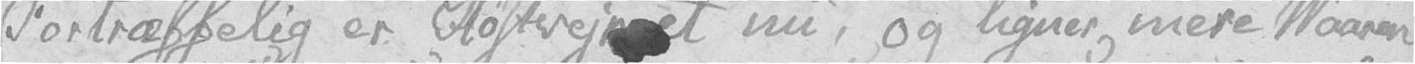Fortræffelig er Høstvejret nu, og ligner mere Vaaren
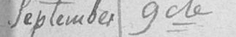September 9de
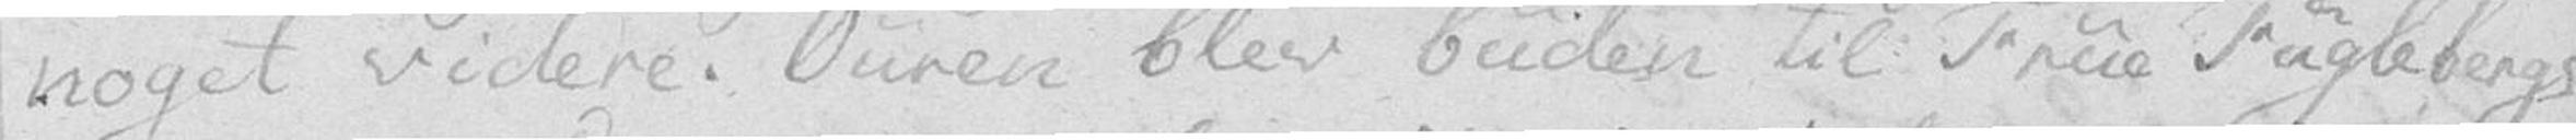noget videre. Ouren blev buden til Frue Fuglebergs
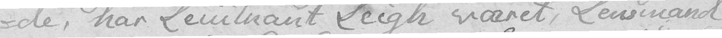-de, har Leiutnant Leigh været, Lensmand
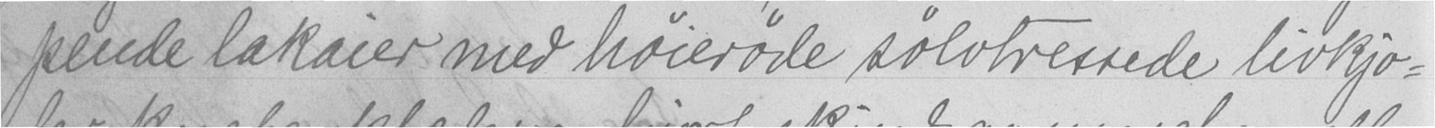pende lakaier med høierøde sølvtressede livkjo-
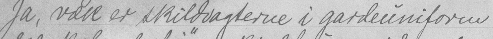Ja, væk er skildvagterne i gardeuniform
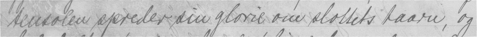tensolen spreder sin glorie om slottets taarn, og
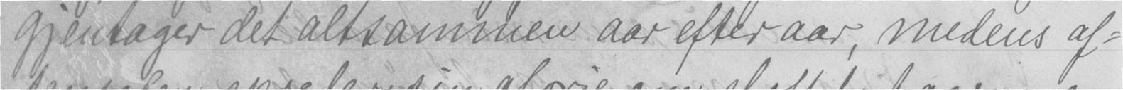gjentager det altsammen aar efter aar, medens af-
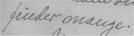finder mange.
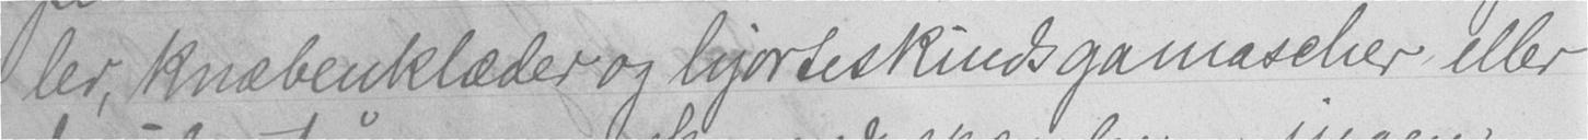ler, knæbenklæder og hjorteskindsgamascher eller
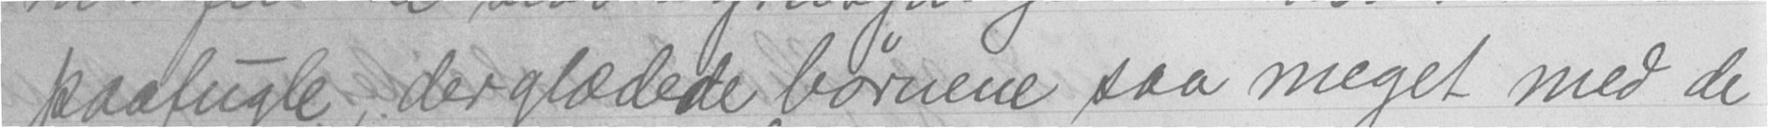paafugle, der glædede børnene saa meget med de
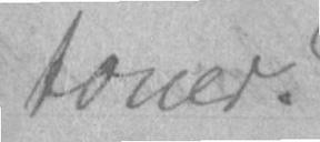toner.
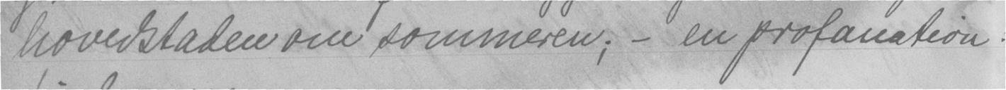hovedstaden om sommeren; - en profanation!
